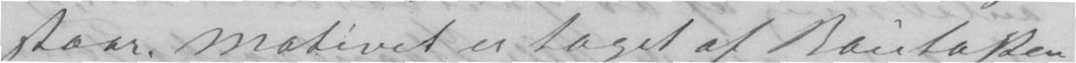staar; motivet er taget af Bautasten,
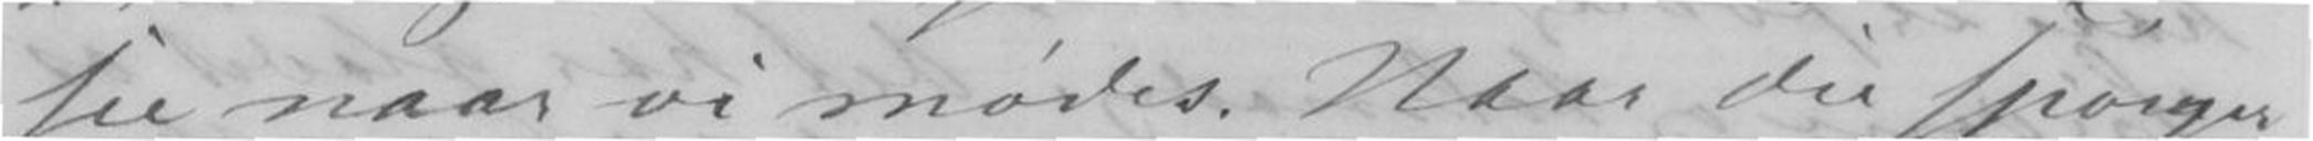see naar vi mødes. Naar du spørger
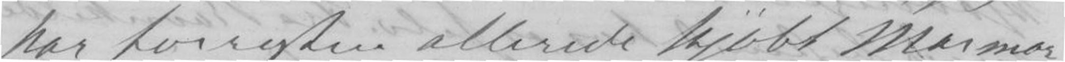har foresten allerede Kjøbt Marmor,
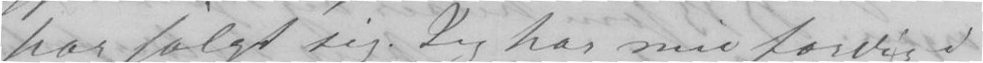har solgt sig. Jeg har nue færdig i
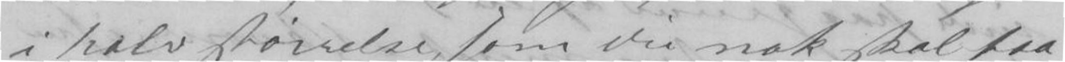i halv størrelse, som du nok skal faa
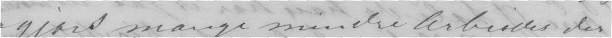gjort mange mindre Arbeider der
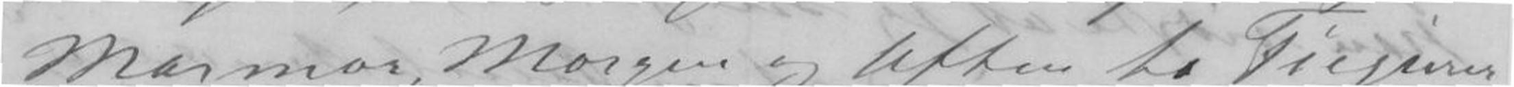Marmor, Morgen og Aften to Figurer
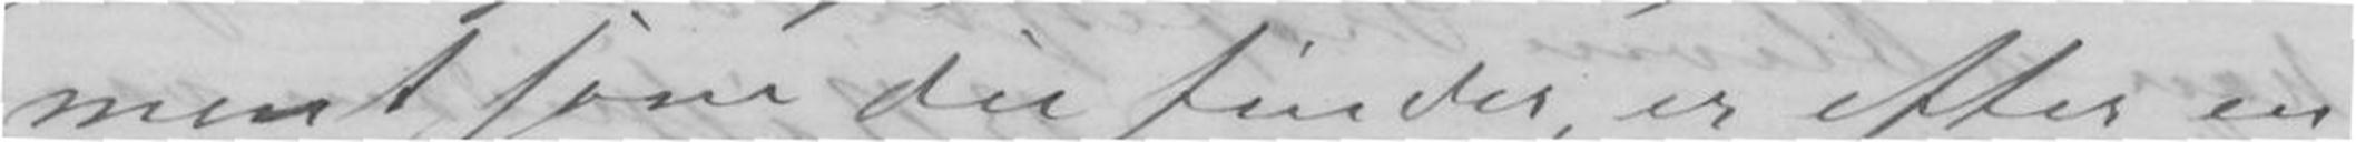ment som du finder, er efter en
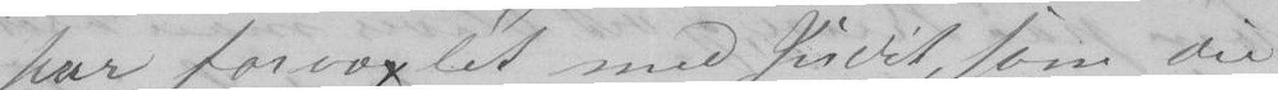har forvexlet med Judit, som du
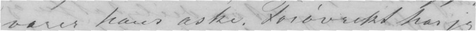varer hans aske. Forøvrikt har jeg
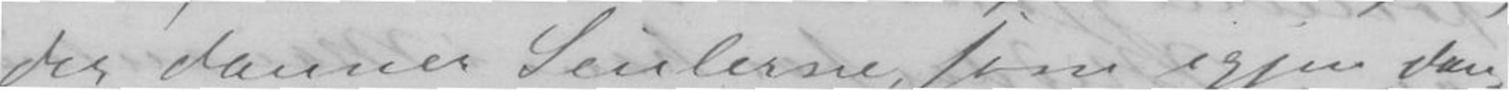der danner Seilerne, som igjen dan-
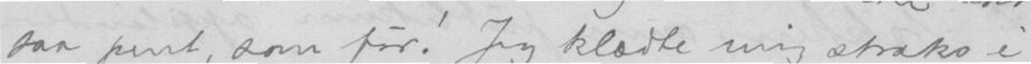saa pent, som før! Jeg klædte mig straks i
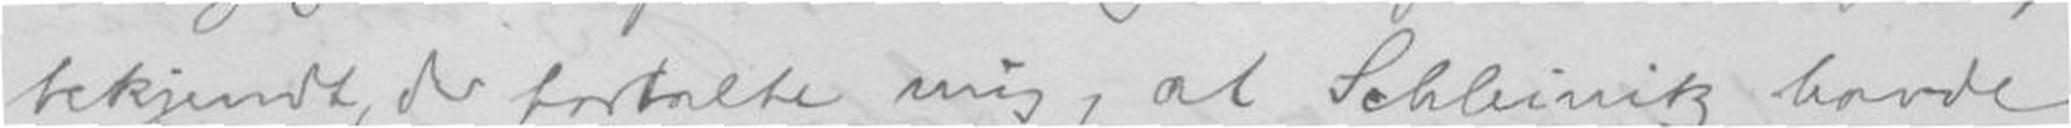bekjendt, de fortalte mig, al Schleinitz havde
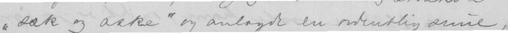"sæk og aske" og anlagde en ordentlig snue
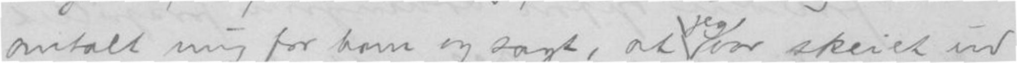omtalt mig for ham og sagt, at jeg var skeiet ud
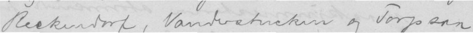Reckendorf, Vanderstucken og Torp saa
